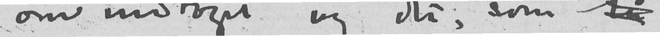om "indtoget" og det, som li
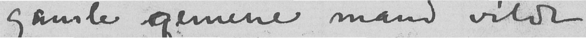gamle gemene mand vilde
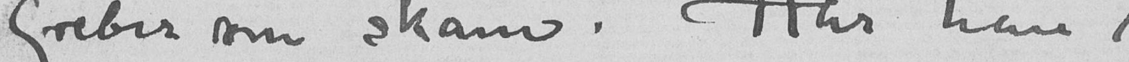greber om skam. Har han
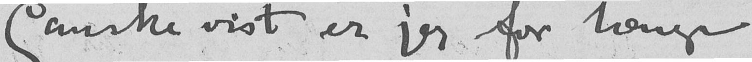Ganske vist er jeg for længe
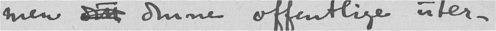men det denne offentlige uter-
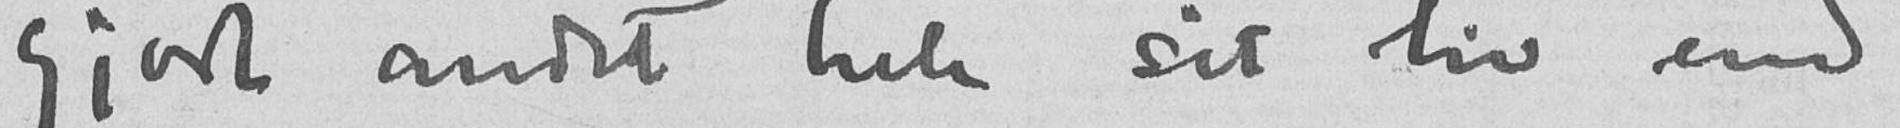gjort andet hele sit liv end
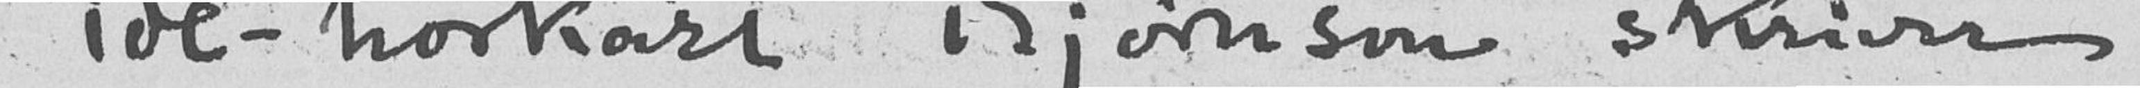idé-horkarl Bjørnson skriver
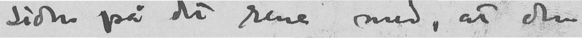siden på det rene med, at den
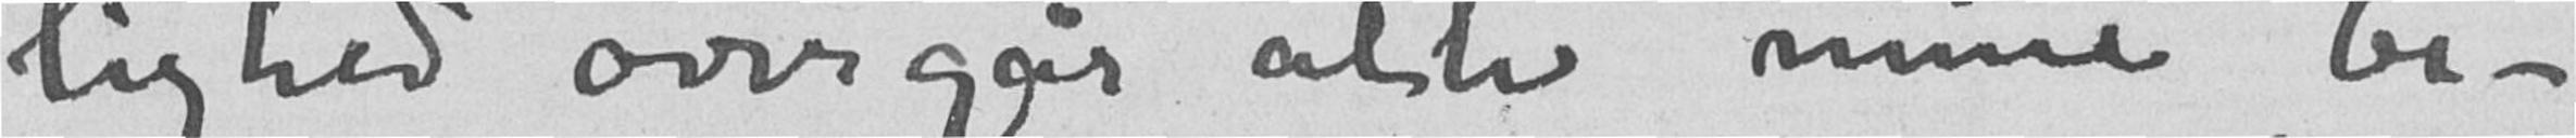lighed overgår alle mine be-
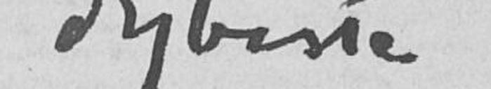dybeste
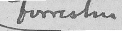forresten
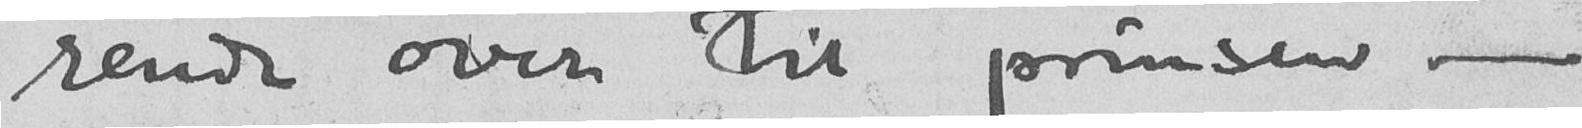rende over Til prinsen -
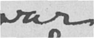var
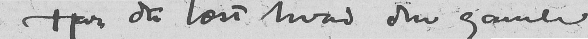Har du læst hvad den gamle
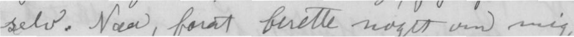selv. Naa, forat berette noget om mig,
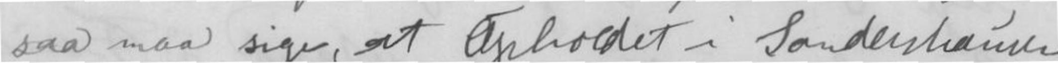saa maa sige, at Opholdet i Sondershausen
